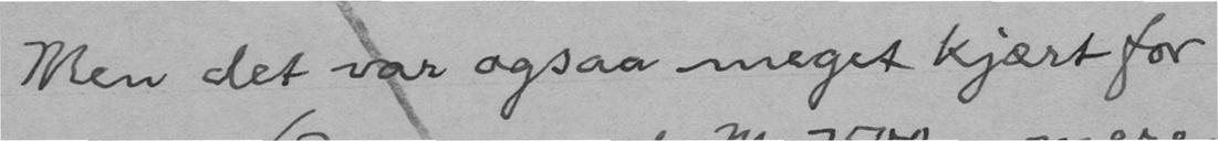Men det var ogsaa meget kjært for
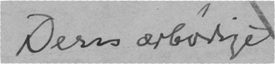Deres ærbødige
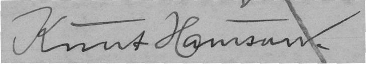Knut Hamsun.
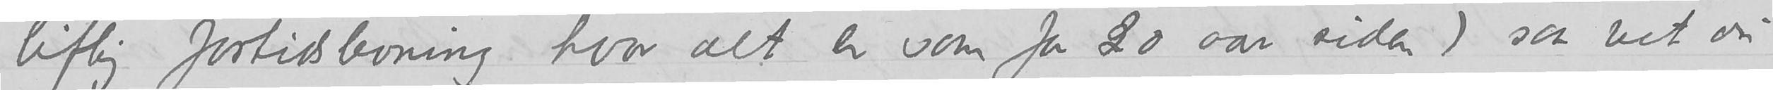billig fortidslevning hvor alt er som for <20> aar siden) saa vet du
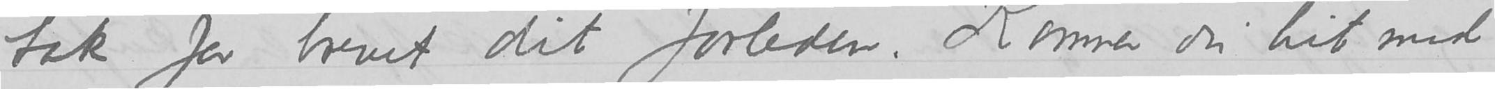tak for brevet dit forleden. Kommer du hit med
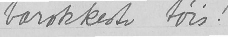barokkeste tøis!
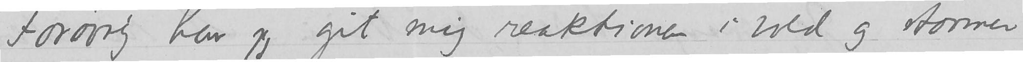Forøvrig har jeg git mig reaktionen i vold og stormern
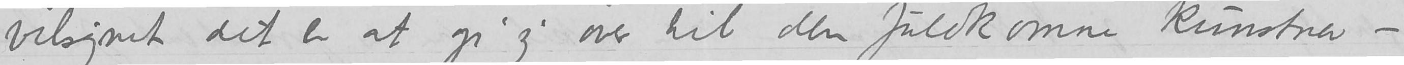velsignet det er at gi sig over til den fuldkomne kunstner –
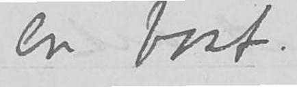en baat.
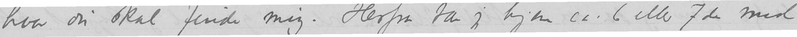hvor du skal finde mig. Herfra tar jeg hjem ca. 6 eller 7de med
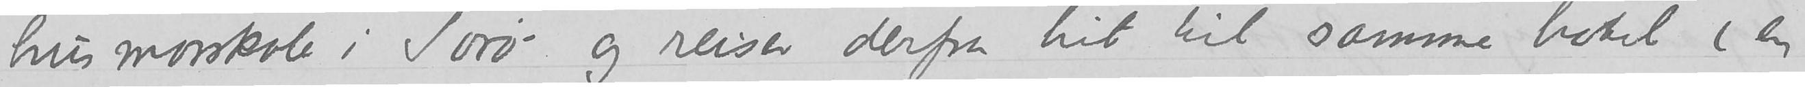husmorskole i Sorø og reiser derfra hit til samme hotel (en
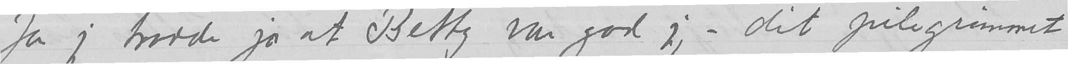Ja jeg troede jo at Betty var god jeg, – dit pilegrimmet
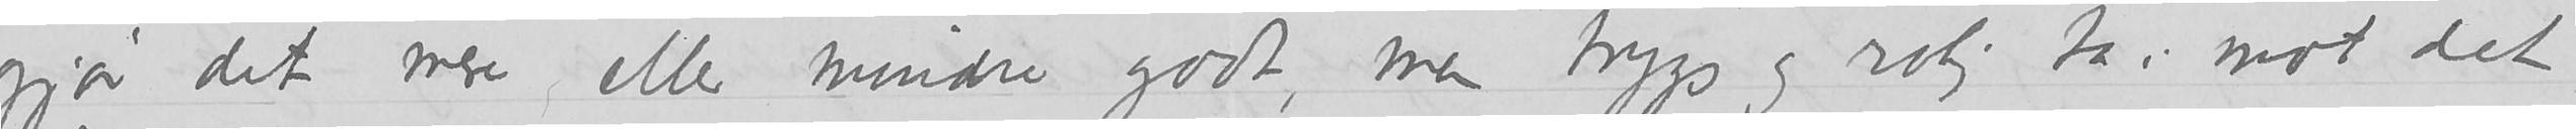gjør det mere eller mindre godt, men trygg og rolig ta i mot det
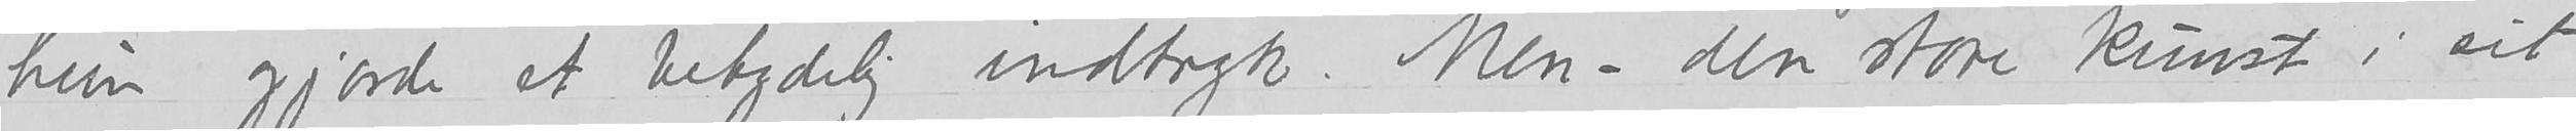hun gjorde et betydelig indtryk. Men – den store kunst i sit
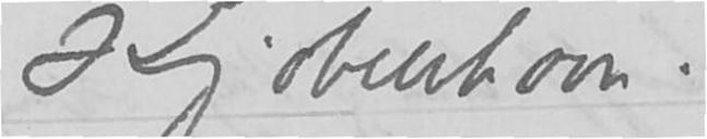Kjøbenhavn.
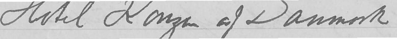Hotel Kongen af Danmark
